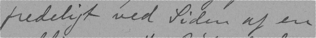fredeligt ved Siden af en
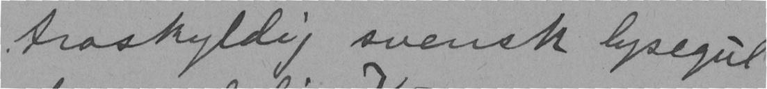troskyldig svensk lysegul
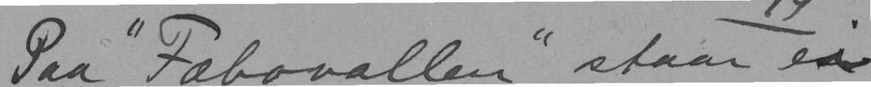Paa "Fæbovallen" staar ei
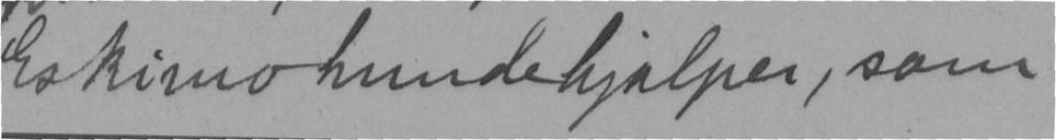Eskimohundehjalper, som
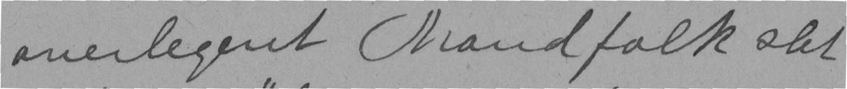overlegent Mandfolk slet
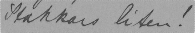Stakkars liten!
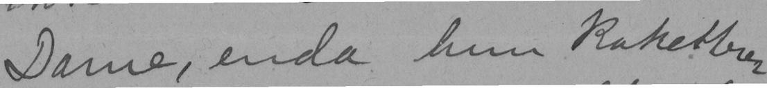Dame, enda hun koketterer
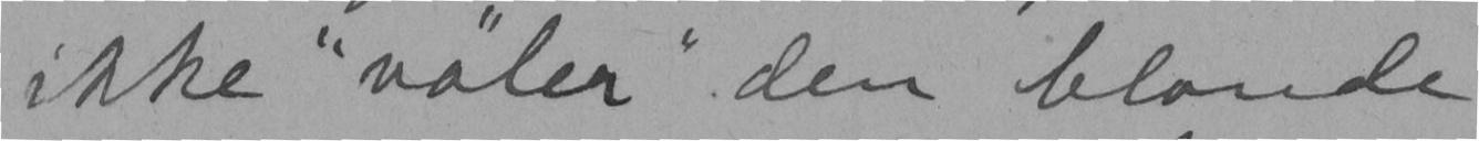ikke "väler" den blonde
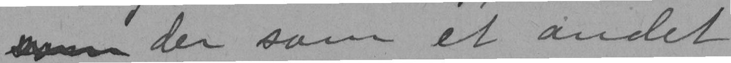der som et andet
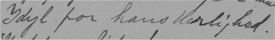Idyl for hans Herlighed.
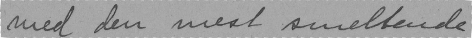med den mest smeltende
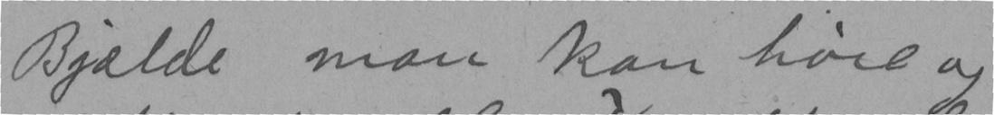Bjælde man kan høre og
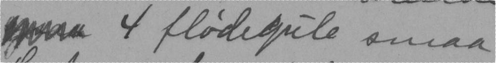4 flødegule smaa
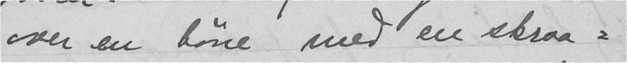over en høne med en skraa-
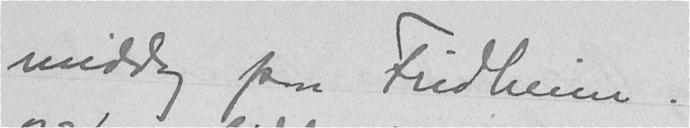middag paa Fridheim.
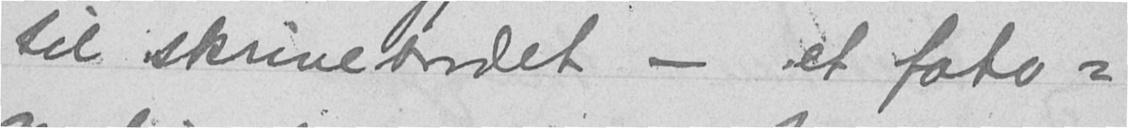til skrivebordet - et foto-
